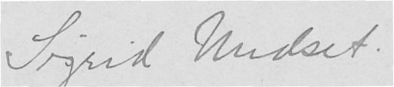Sigrid Undset.
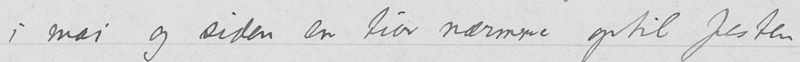i mai og siden en tur nærmere optil festen
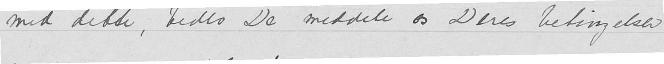med dette, bedes De meddele os Deres betingelser
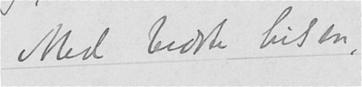Med bedste hilsen,
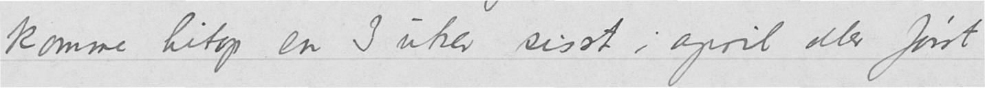komme hitop en 3 uker sisst i april eller først
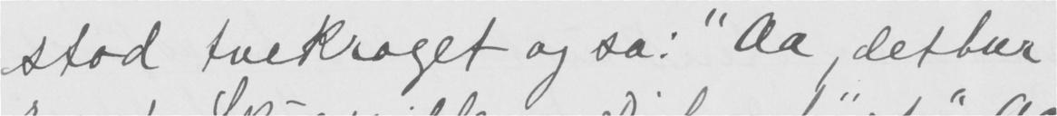stod tvekroget og sa: «Aa det har
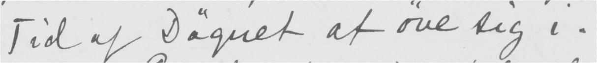Tid af Døgnet at øve sig i.
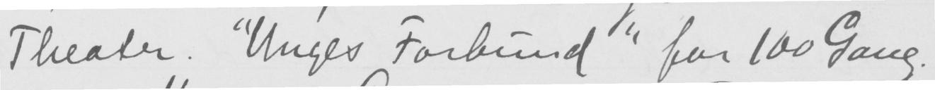Theater. «Unges Forbund» for 100 Gang.
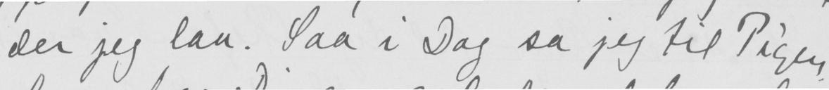der jeg laa. Saa i Dag sa jeg til Pigen,
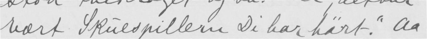vært Skuespillern Di har hørt.» Aa
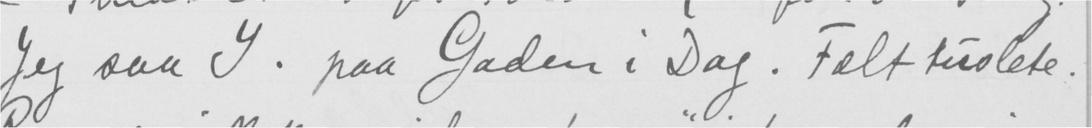Jeg saa Y. paa Gaden i Dag. Fælt tuslete.
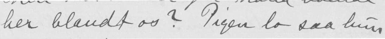her blandt os? Pigen lo saa hun
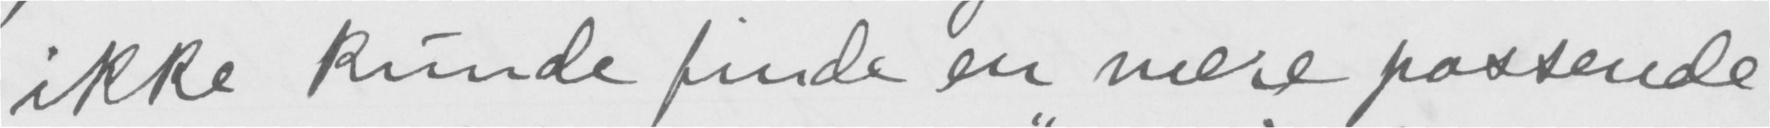ikke kunde finde en mere passende
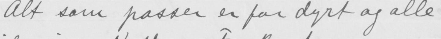Alt som passer er for dyrt og alle
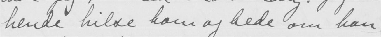hende hilse ham og bede om han
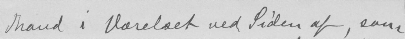Mand i Værelset ved Siden af, som
